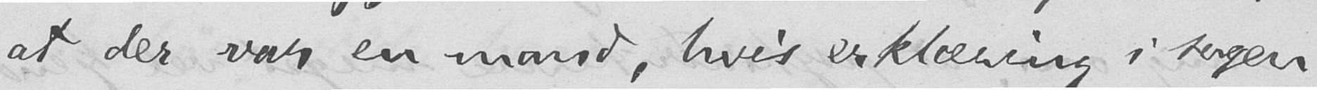at der var en mand, hvis erklæring i sagen
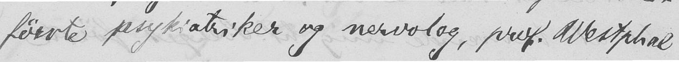første psykiatriker og nervolog, prof. Westphal
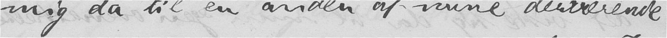mig da til en anden af mine derværende
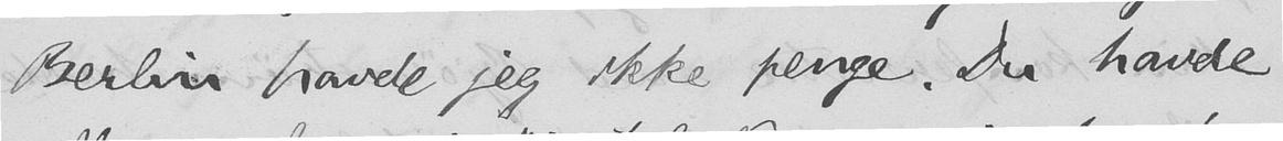Berlin havde jeg ikke penge. Du havde
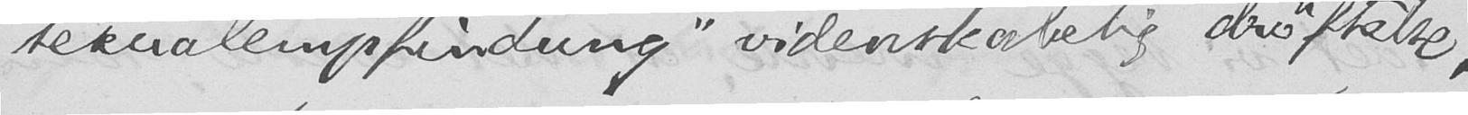sexualempfindung" videnskabelig drøftelse,
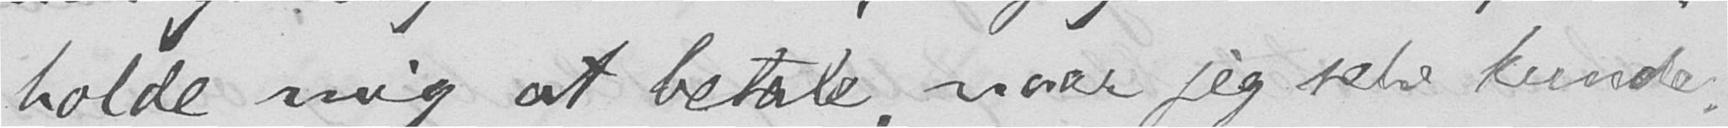holde mig at betale, naar jeg selv kunde.
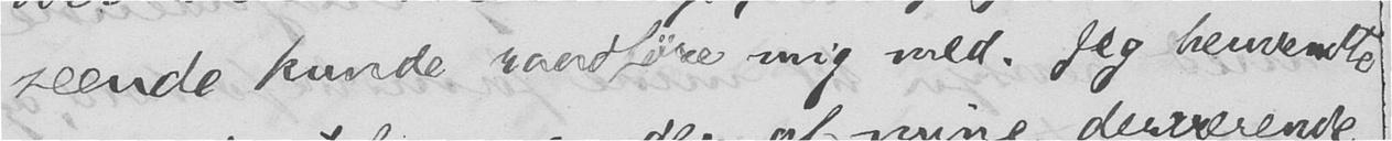seende kunde raadføre mig med. Jeg henvendte
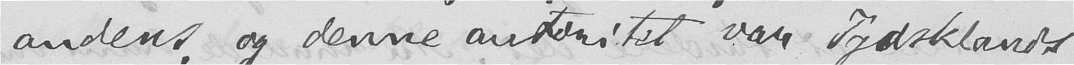andens, og denne autoritet var Tydsklands
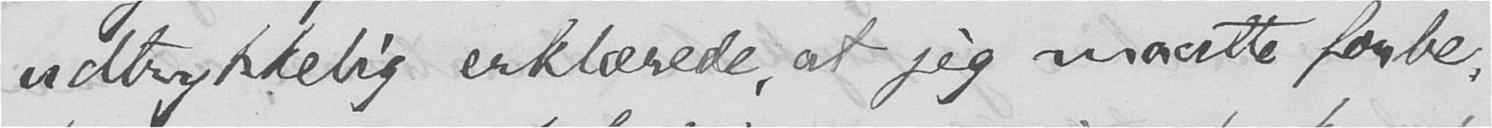udtrykkelig erklærede, at jeg maatte forbe-
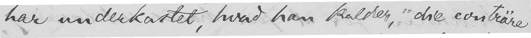har underkastet, hvad han kalder, "die conträre
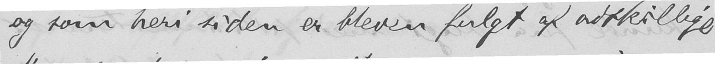og som heri siden er bleven fulgt af adskillige.
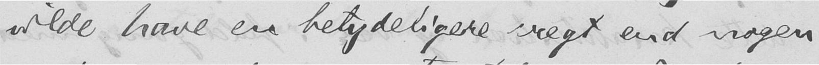vilde have en betydeligere vægt end nogen
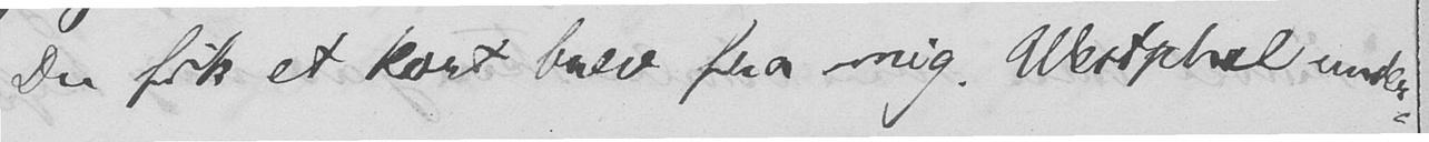Du fik et kort brev fra mig. Westphal under-
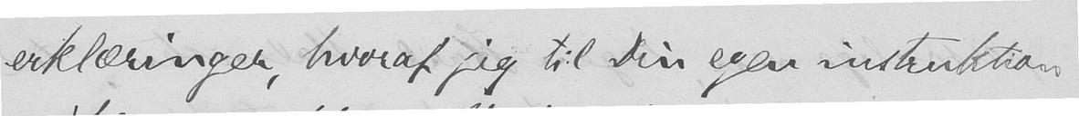erklæringer, hvoraf jeg til Din egen instruktion
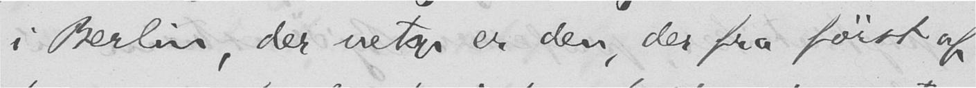i Berlin, der netop er den, der fra først af
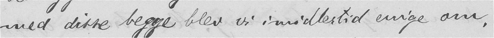med disse begge blev vi imidlertid enige om,
